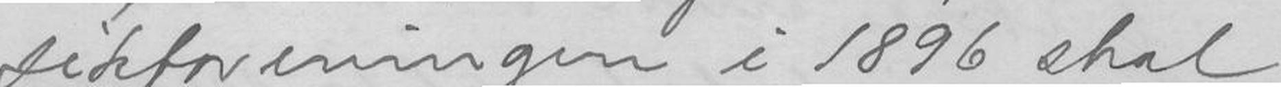sikkforeningen i 1896 skal
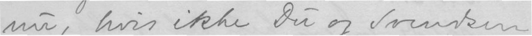nu, hvis ikke Du og Svendsen
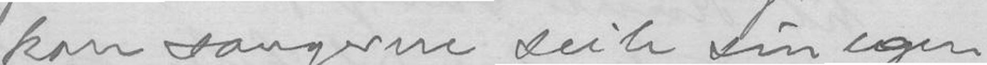kan sagerne seile sin egen
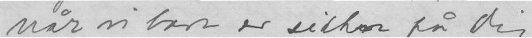når vi bare er sikker på dig
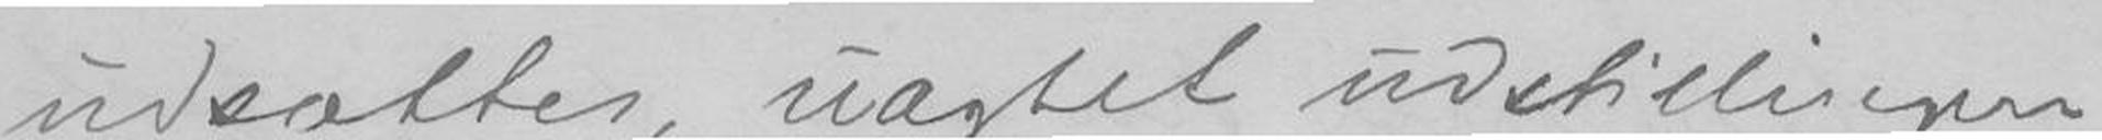udsættes, uagtet udstillingen
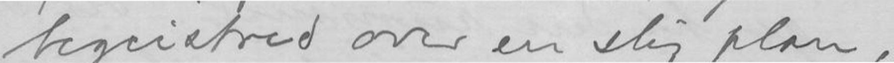begeistred over en slig plan,
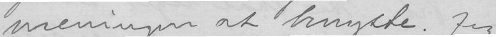meningen at benytte Jeg
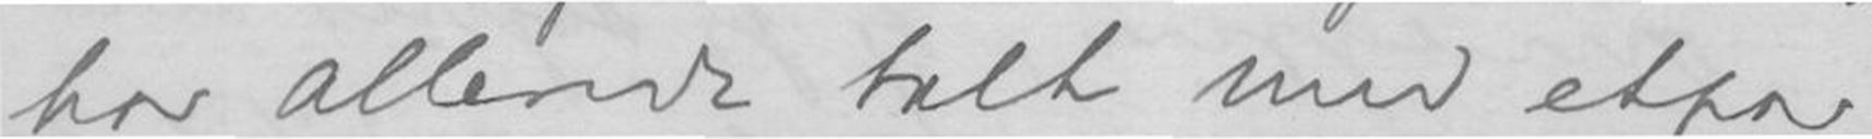har allerede talt med etpar
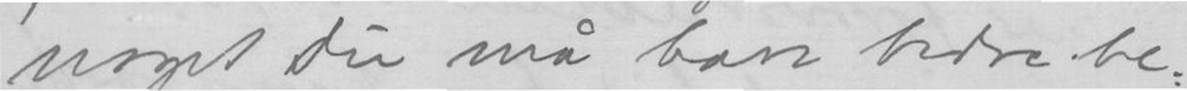noget du må have bedre be-
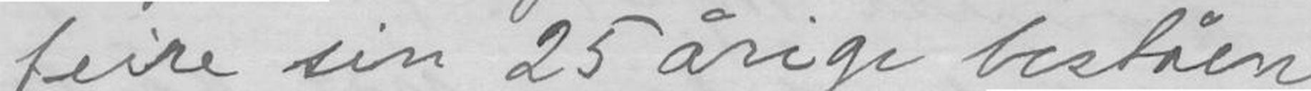feire sin 25 årige beståen
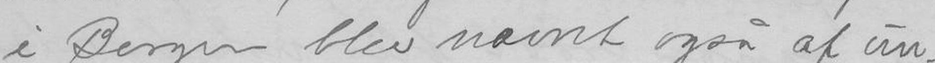i Bergen blev nævnt også af un
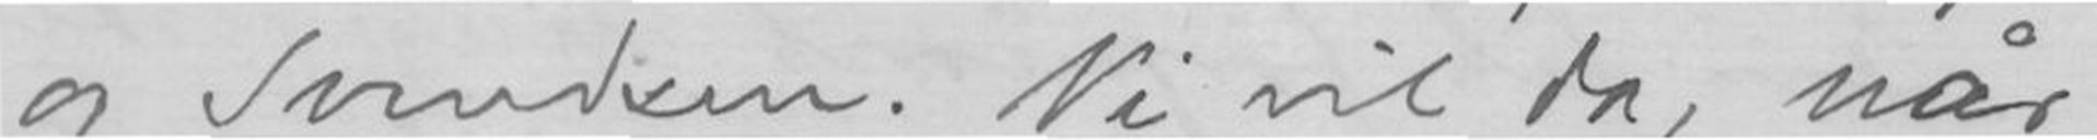og Svendsen. Vi vil da, når
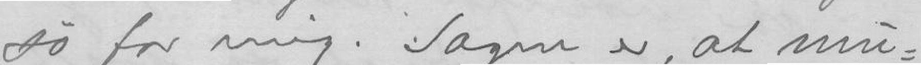sø for mig. Sagen er, at mu-
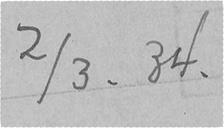2/3. 34.
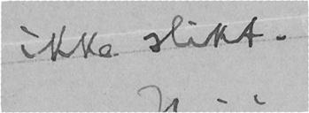ikke slikt.
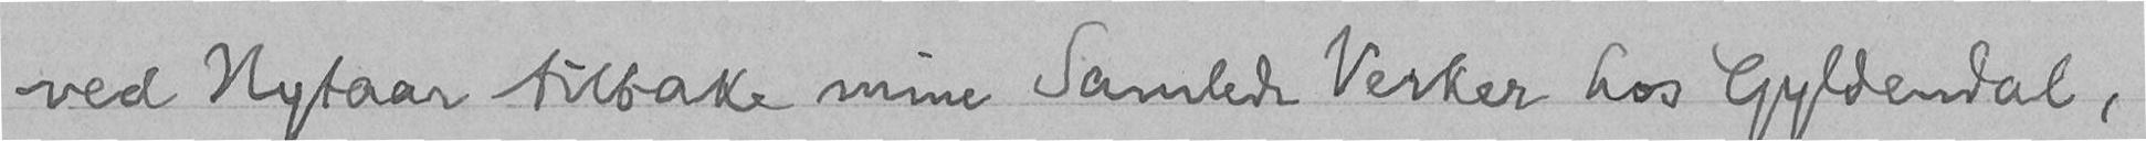ved Nytaar tilbake mine Samlede Verker hos Gyldendal,
